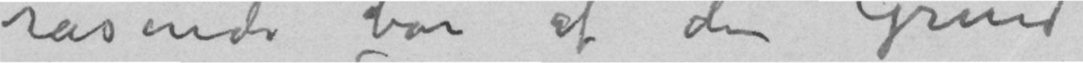rasende bare af den Grund
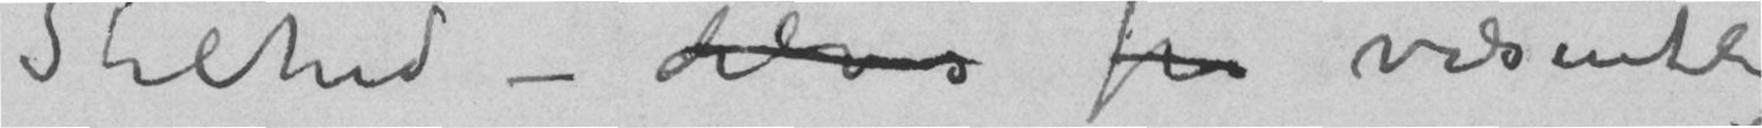Stilhed – delvis fra væsentlig
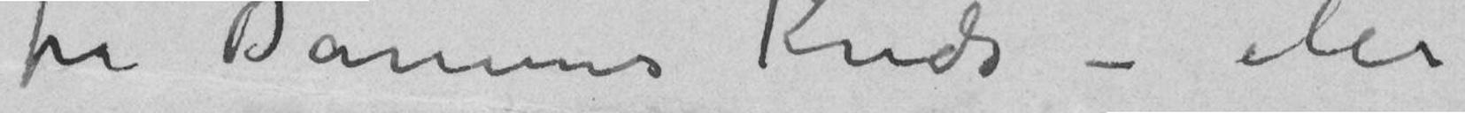fra Damens Kreds – Men
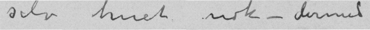selv truet nok – dermed
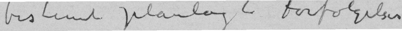bestemt planlagt Forfølgelse
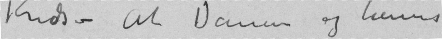Kreds – At Damen og hennes
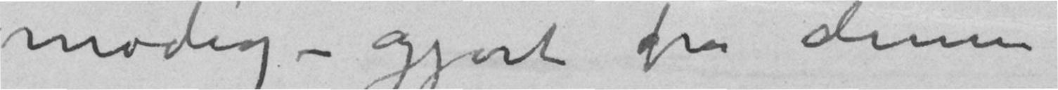modig – gjort fra denne
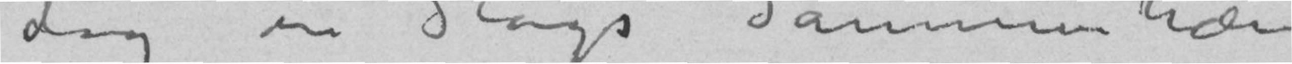dog en Slags Sammenhæng
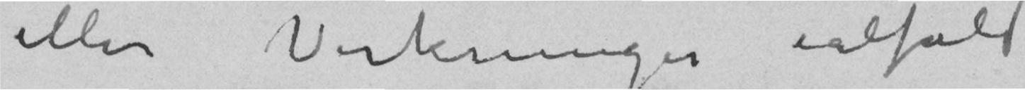eller Virkninger ialfald
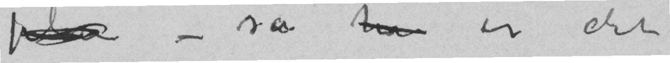– så har er det
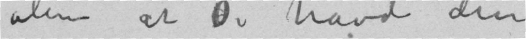alene at de havde den
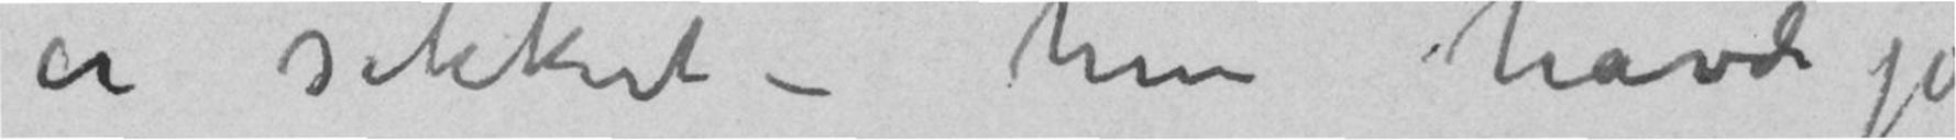er sikkert – hun havde jo
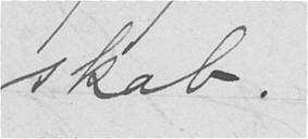skab.
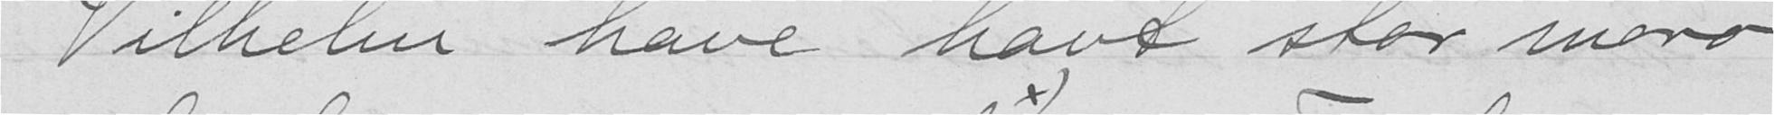Vilhelm have havt stor moro
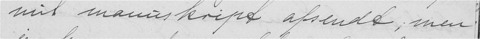mit manuskript afsendt; men
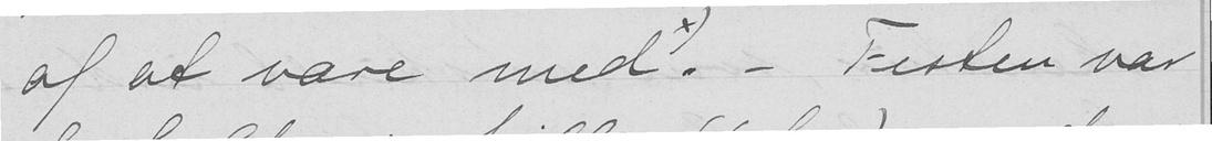af at være med x). - Festen var
